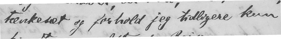tænkesæt og forhold jeg tidligere kun
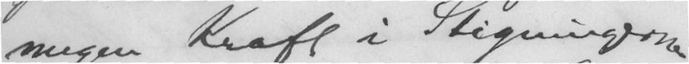megen Kraft i Stigningerne
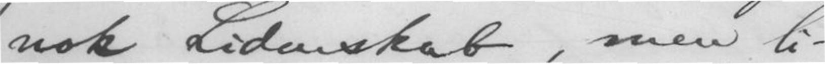nok Lidenskab, men li-
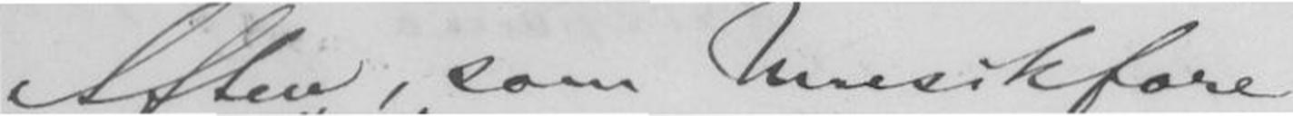Aften, som Musikfore-
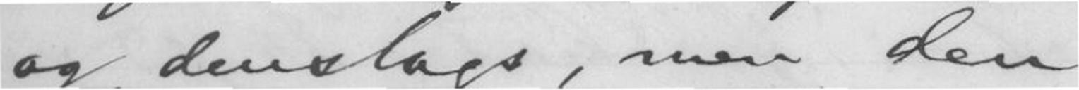og denslags, men den
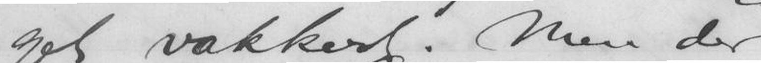get vakkert. Men der
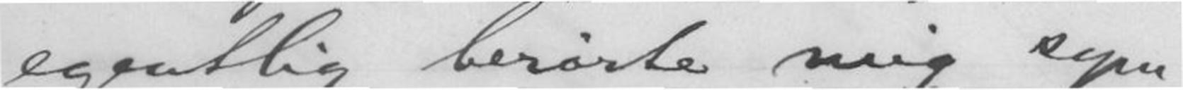egentlig berørte mig sym-
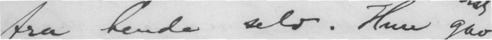fra hende selv. Hun gav
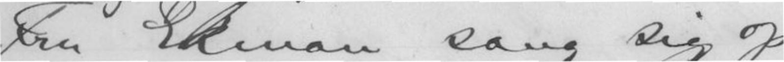Fru Ekman sang sig op
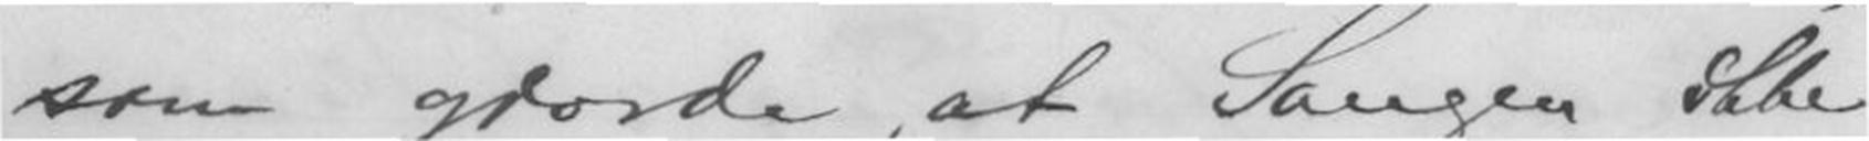som gjorde, at Sangen ikke
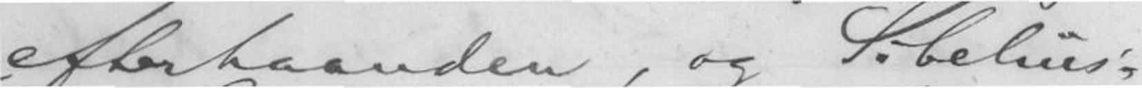efterhaanden, og Sibelius's
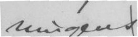blev baade M. og jeg tem-
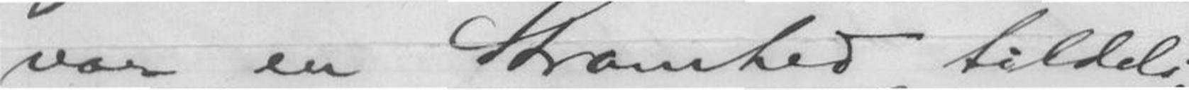var en Stramhed tildels,
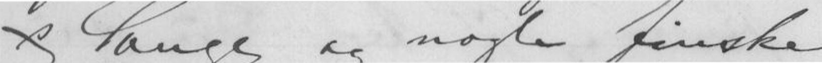s Sange og nogle finske
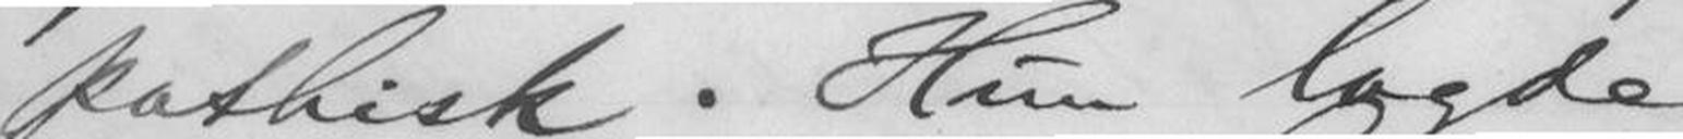pathisk. Hun lagde
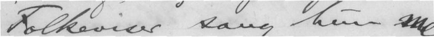Folkeviser sang hun me-
